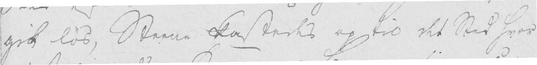gik løs, Steene kastedes og til det sted hvor
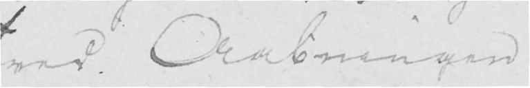ved Aabningen
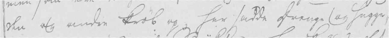den og andre krøb og her sadde Drenge og hugge-
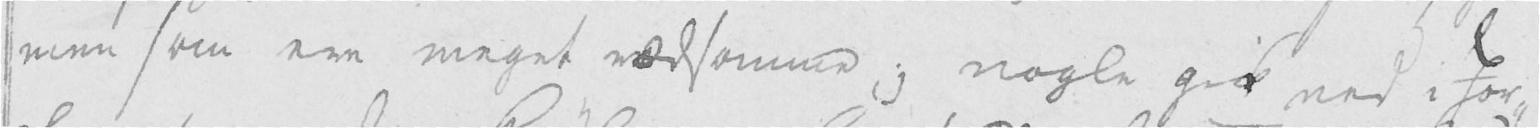men som ere meget rædsomme; nogle gik ned i Jor-
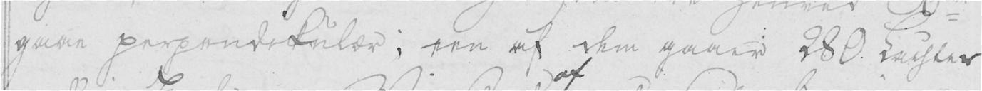gaae perpendikulær; een af dem gaaer 280 Lachter
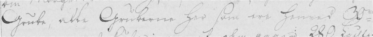Grube, alle Gruberne her som ere henved 30 e
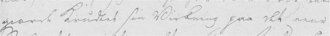giorde Krudtet sin Virkning paa det eene
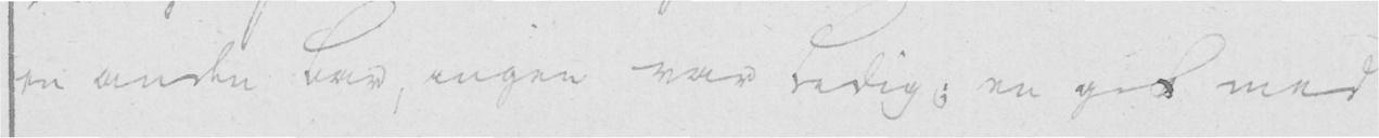en anden bar, ingen var ledig, en gik med
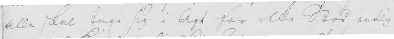alle skal tage sig i Agt for dette Stæd, endlig
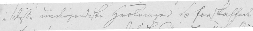i disse underjordiske Hvælvinger og forskaffede
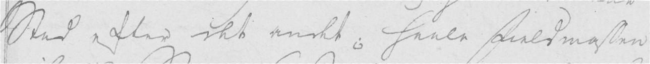Sted efter det andet; Heele Fieldmassen
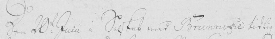Den 20de Julii i Selskab med Brunnicke tidlig
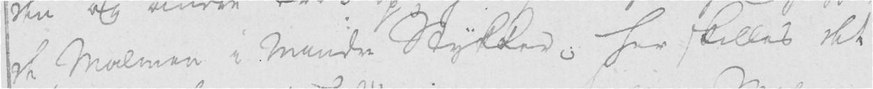de Malmen i mindre Stykker; her skilles det
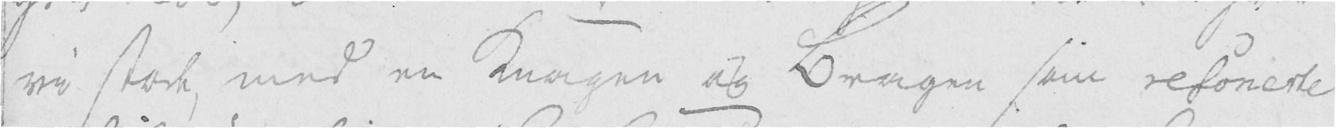vi stode, med en Knagen og Bragen som resonerte
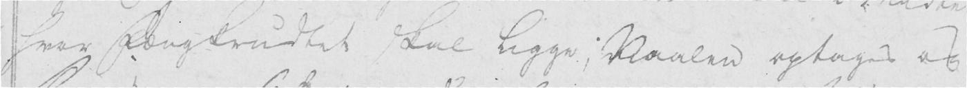hvor Fængkrudtet skal ligge; Naalen optages og
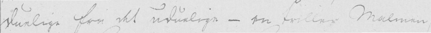duelige fra det uduelige - en triller Malmen,
